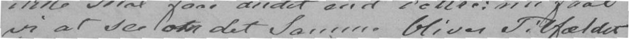vi at see om det Samme bliver Tilfældet
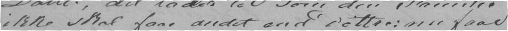ikke skal faae andet end Døttre: nu faae
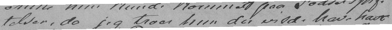telsen, da jeg troer hun der vilde have havt
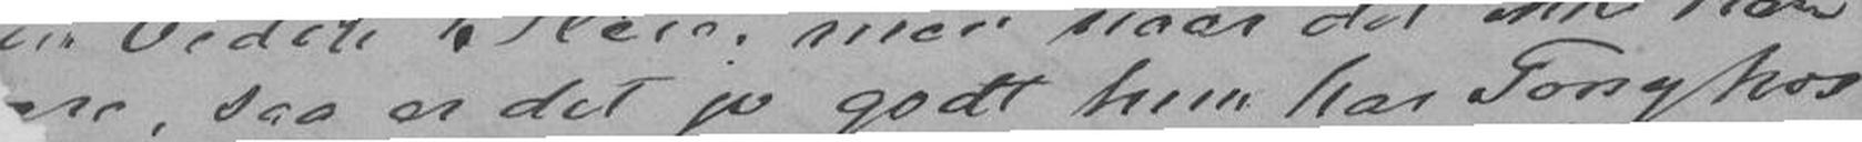, saa er det jo godt hun har Tony hos
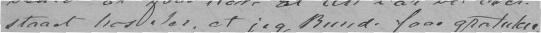staaet hos Jer, at jeg kunde faae gratulere,
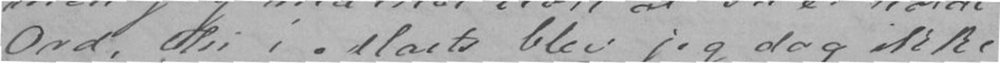Ord, thi i Marts blev jeg dog ikke
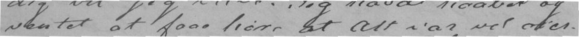ventet at faae høre at Alt var vel over-
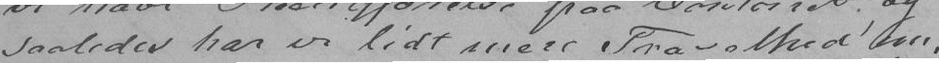saledes har vi lidt mere Travelhed nu,
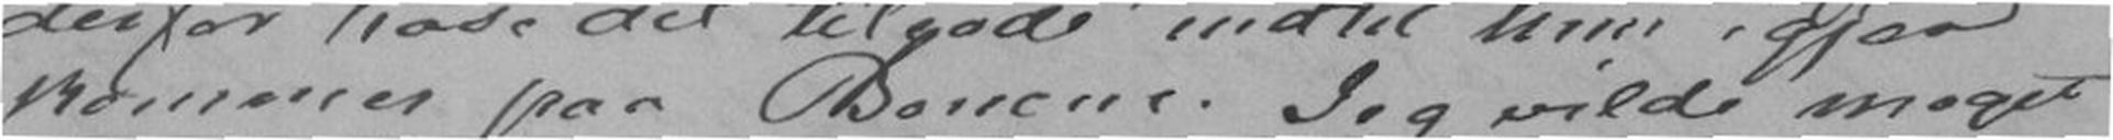kommer paa Benene. Jeg vilde meget
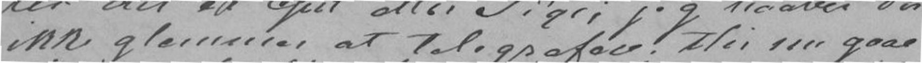ikke glemmer at telegrafere. Thi nu gaae
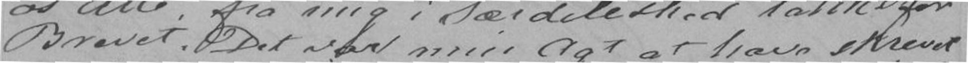Brevet. Det var min Agt at have skrevet
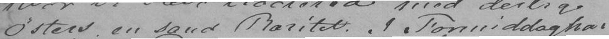Østers, en sand Raritet. I Formiddag har
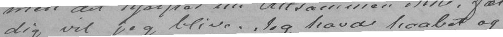dig vil jeg blive. Jeg havde haabet og
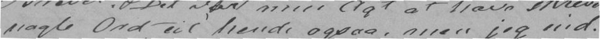nogle Ord til hende ogsaa, men jeg ind-
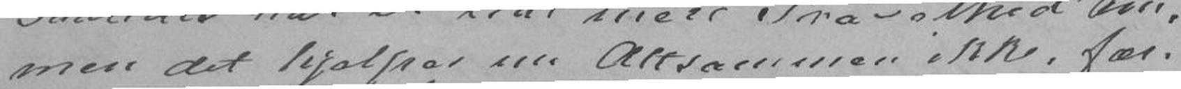men det hjælper nu Altsammen ikke, fær-
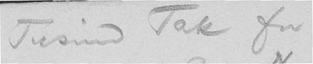Tusind Tak for
